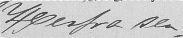Herfra sen-
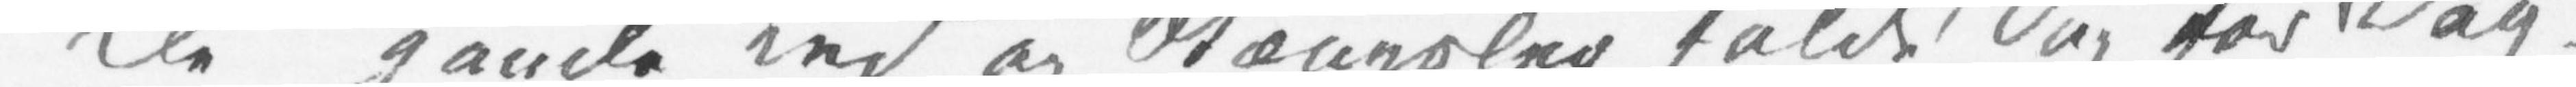De gamle Led og Stængsler falde Dag for Dag.
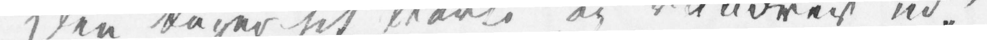Den tager sit Parti og vandrer ud!
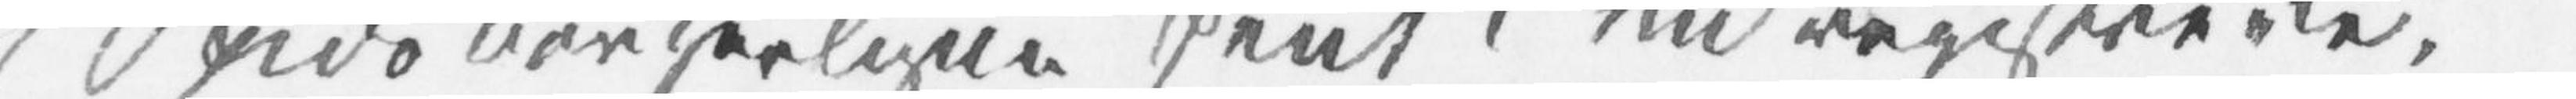Spidsborgerligen pent indregistrere,
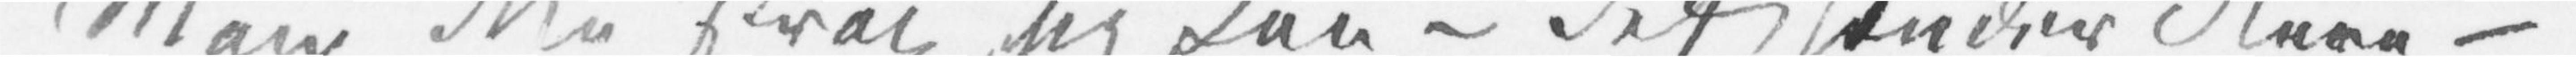Man ikke strax sig kan – det hænder Flere –
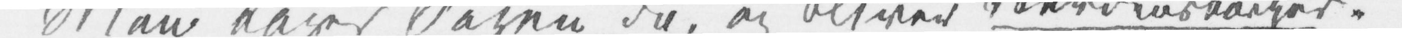Man tager Sagen da, og bliver Verdensborger.
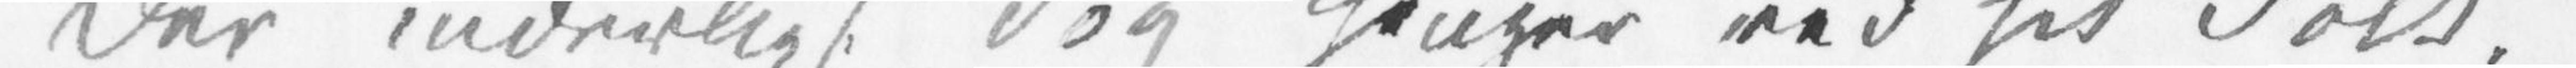Der inderligt dog henger ved sit Folk.
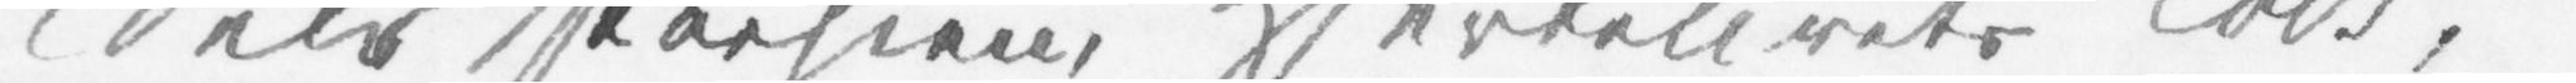Selv Poesien, Hjertelivets Tolk,
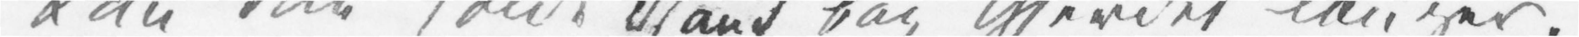Kan ikke holde Stand bag Gjerdet længer.
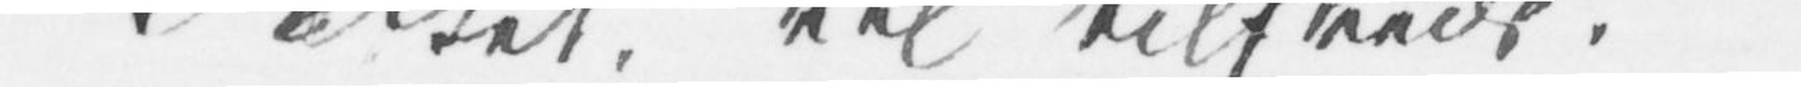Fattet, vel tilfreds.
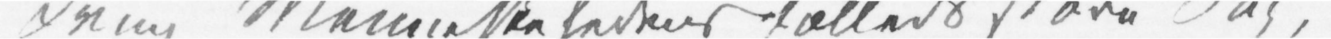Kring Menneskehedens fælleds store Sag,
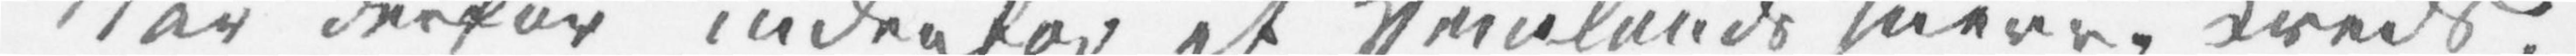.. Når derfor indenfor et Hjemlands snevre Kreds,
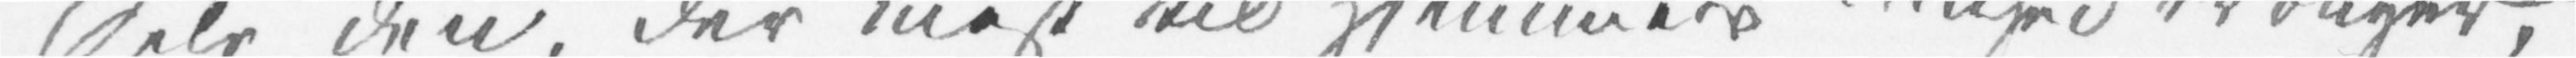Selv den, der mest til Hjemmets Lunhed trænger,
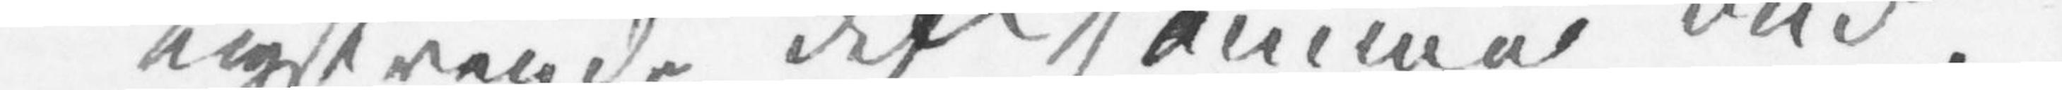Lystrende det samme Bud,
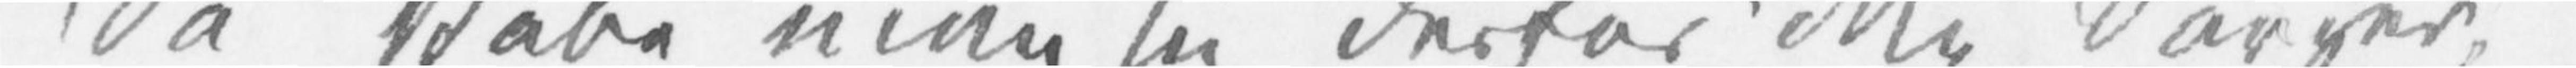Så skabe man sig derfor ikke Sorger,
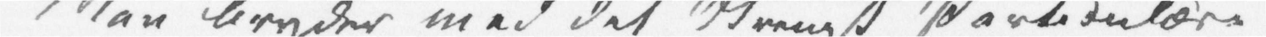Man bryder med det strengt Partikulære
